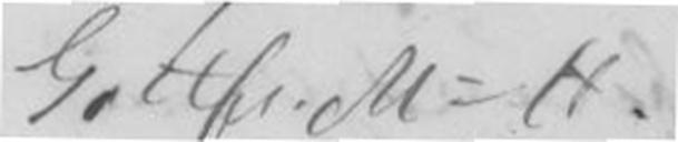Gottfr. M-H.
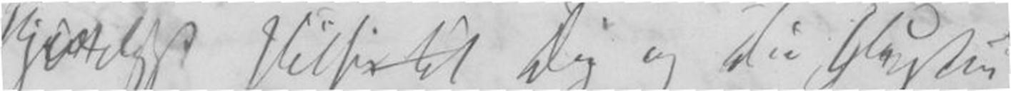Hjærteligst Hilsen til Dig og din Hustru
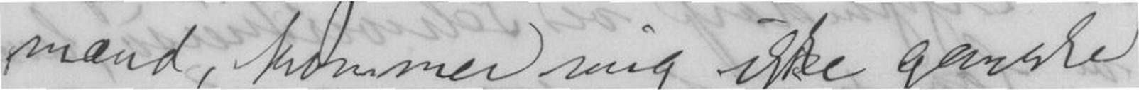mænd, kommer mig ikke ganske
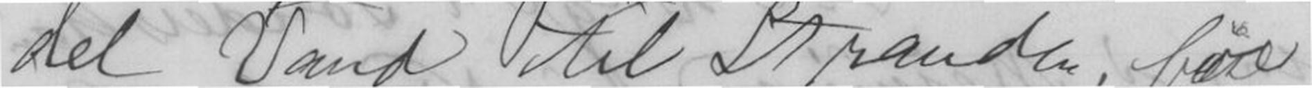del Vand til Stranden, før
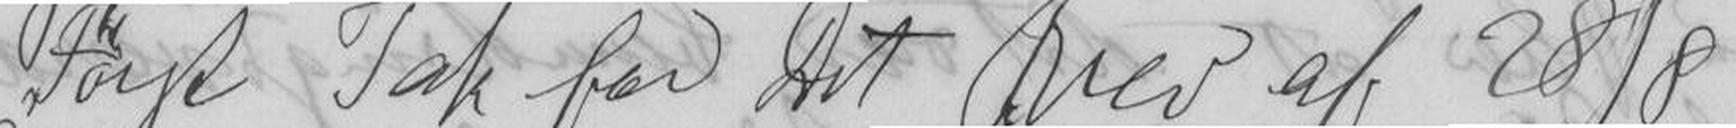Først Tak for Dit Brev af 28/8 !
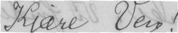Kjære Ven !
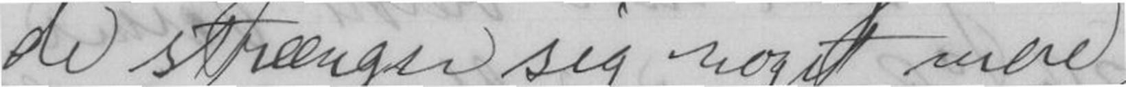de strænger sig noget mere
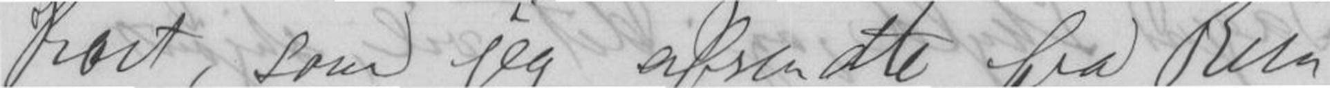kort, som jeg afsendte fra Bern
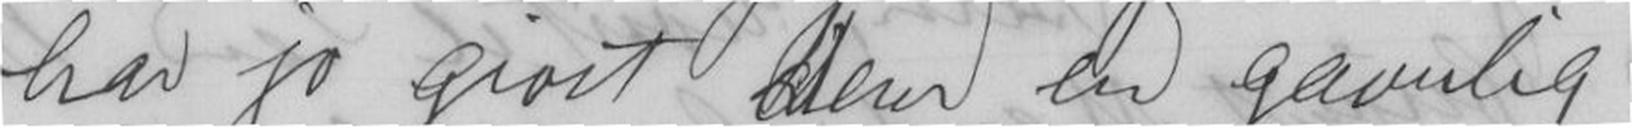har jo givet dem en gavnlig
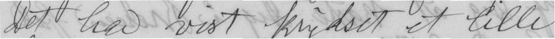Det har vist krydset et lille
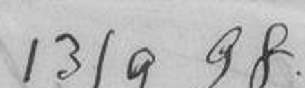13/9 98.
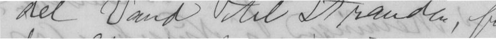sidst dog have til Følge, at
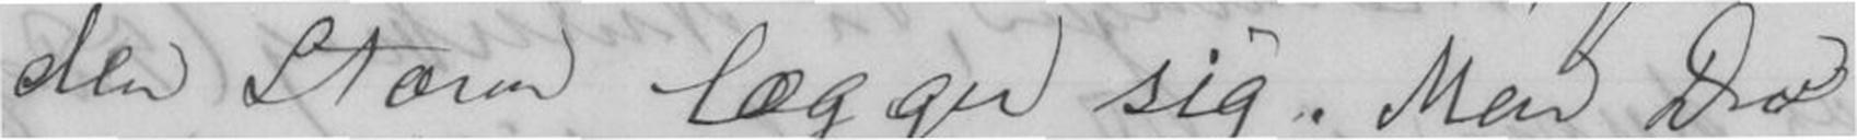den Storm lægger sig. Men Du
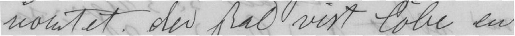uvæntet der skal vist løbe en
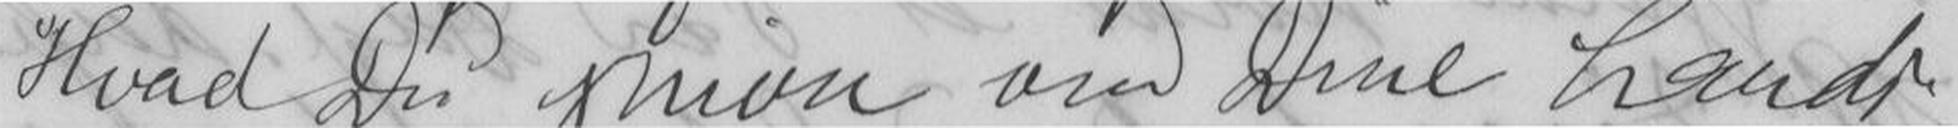Hvad Du skriver om Dine Lands-
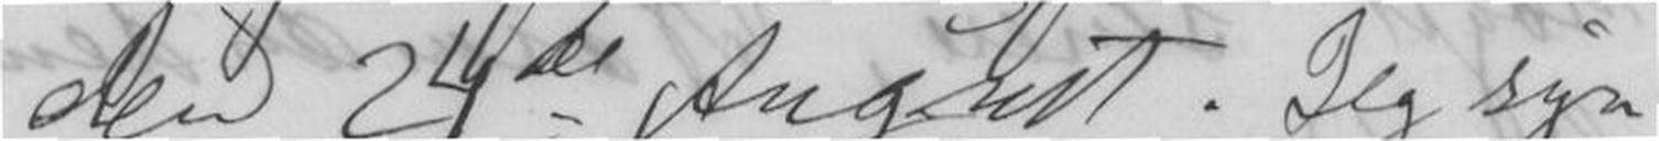den 24de August. Jeg syn-
